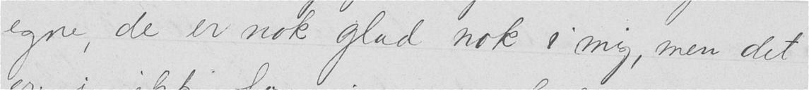egne, de er nok glad nok i mig, men det
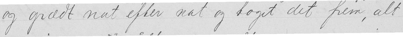og grædt nat efter nat og taget det frem, alt
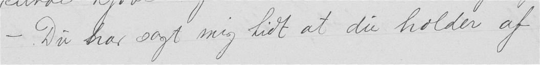- Du har sagt mig tidt at du holder af
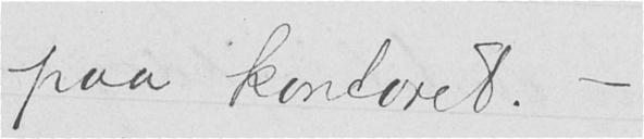paa kontoret. -
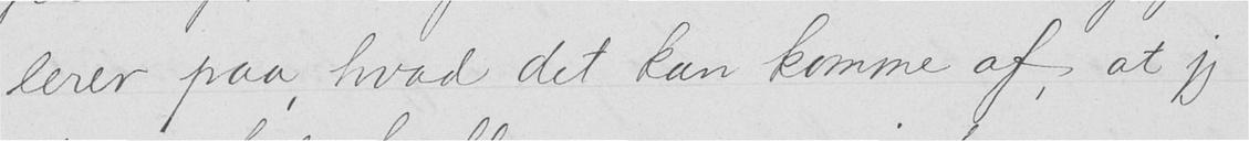lerer paa, hvad det kan komme af, at jeg
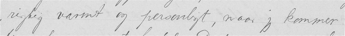rigtig varmt og personligt, naar jeg kommer
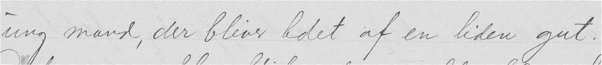ung mand, der bliver ledet af en liden gut.
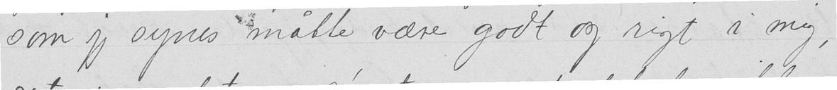som jeg synes maatte være godt og rigt i mig,
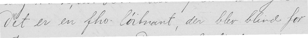Det er en fhv. løitnant, der blev blind for
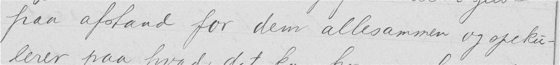paa afstand for dem allesammen og speku-
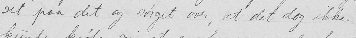set paa det og sørget over, at det dog ikke
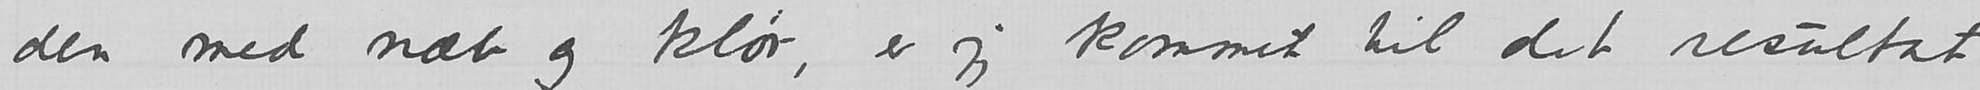den med næb og klør, er jeg kommet til det resultat,
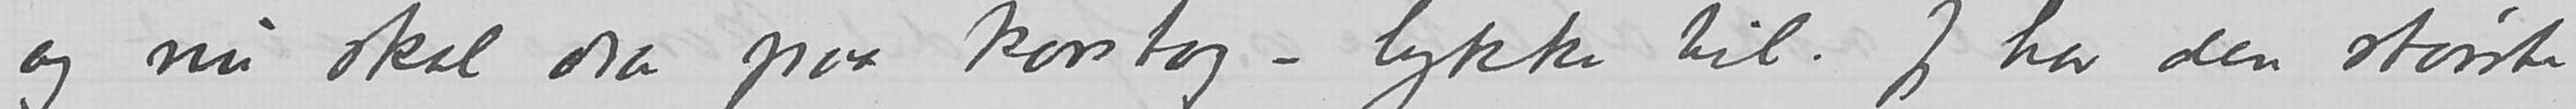og nu skal dra paa korstog - lykke til. Jeg har den største
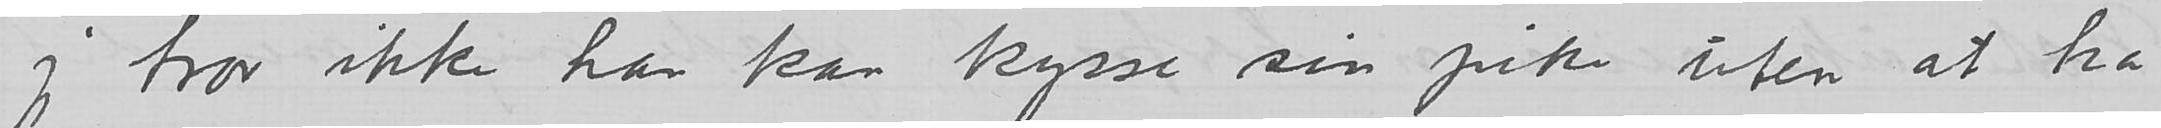jeg tror ikke han kan kysse sin pike uten at ha
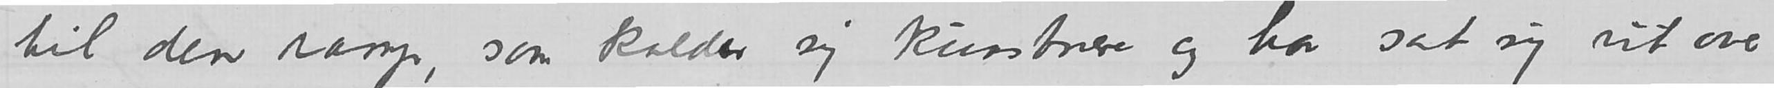til den ramp, som kaller sig kunstnere og har sat sig
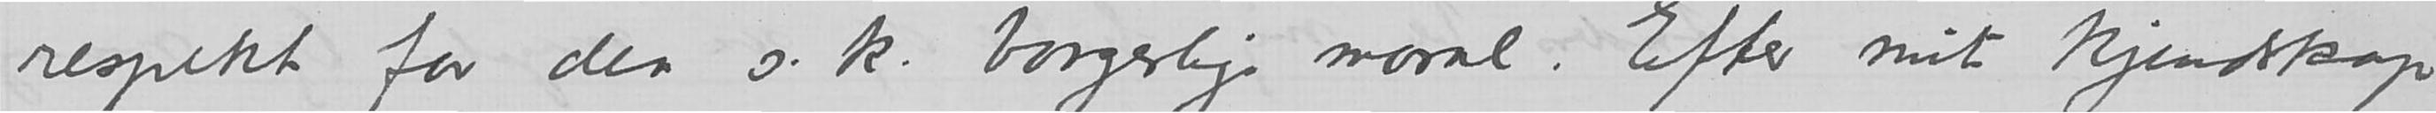respekt for den s.k. borgerlige moral. Efter mit kjendskap
